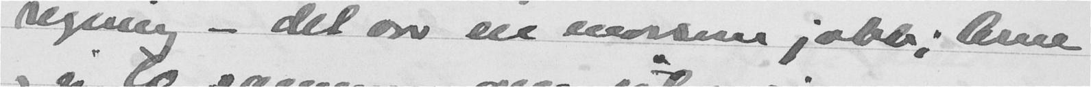regning - det var en morsom jobb; Arne
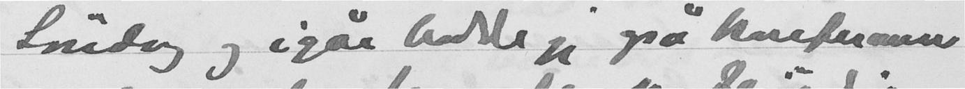Lørdag og igår hadde jeg også konferanse
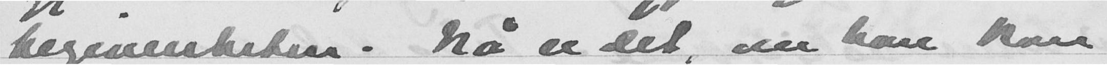begivenheten. Nå er det, om han kan
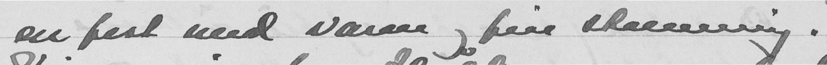en fest med varm og fin stemning.
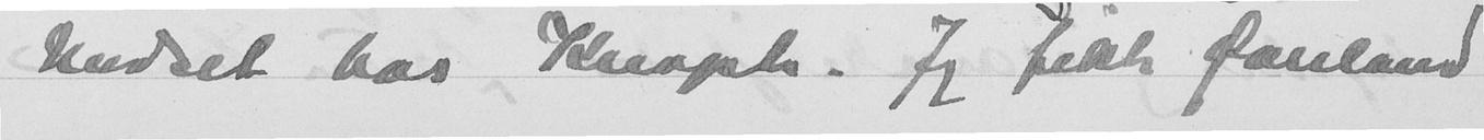Undset hos Knoph. Jeg fikk fanland
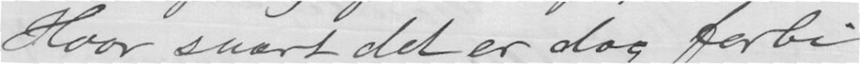Hvor snart det er dog forbi
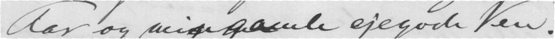Far og min gamle ejegode Ven.
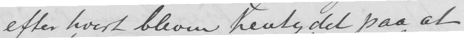efter hvert bleven hentydet paa at
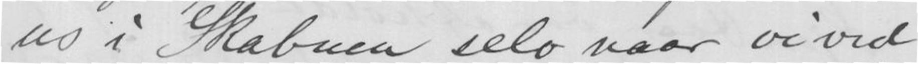os i Skæbnen selv naar vi ved
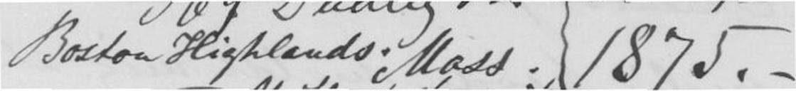Boston Highlands. Mass. |1875. -
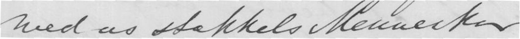med os stakkels Mennesker
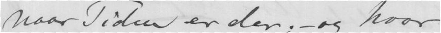naar Tiden er der; - og hvor
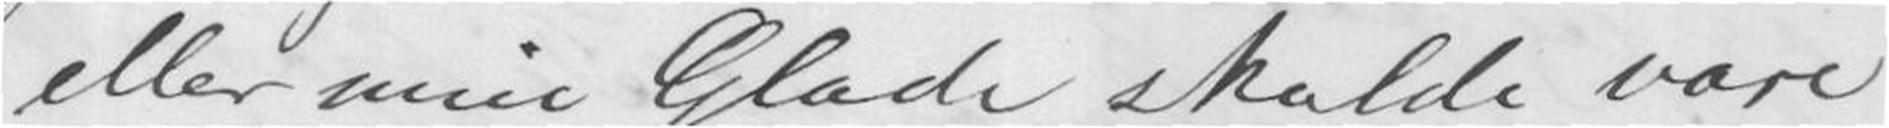eller min Glæde skulde vare
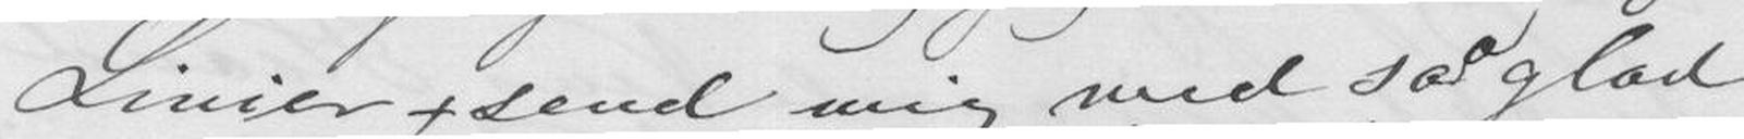Linier send mig med saa glad
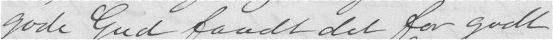gode Gud fandt det for godt
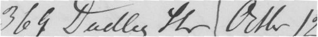369 Dadley Str|Octbr 12 th
